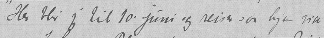Her blir jeg til 10. juni og reiser saa hjem via
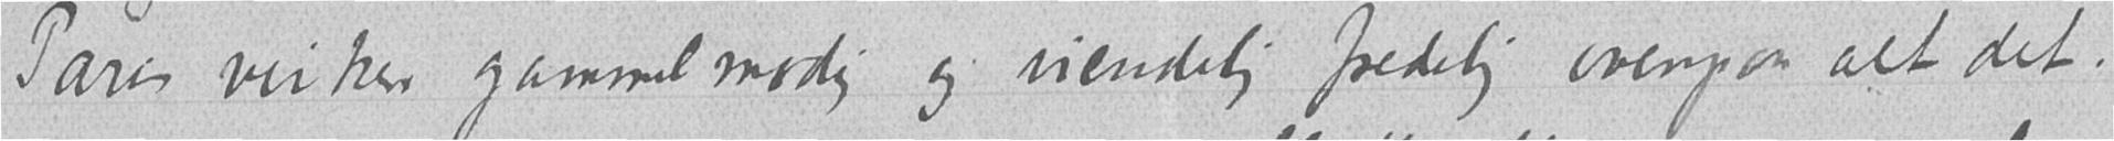Paris virker gammelmodig og uendelig fredelig ovenpaa alt det.
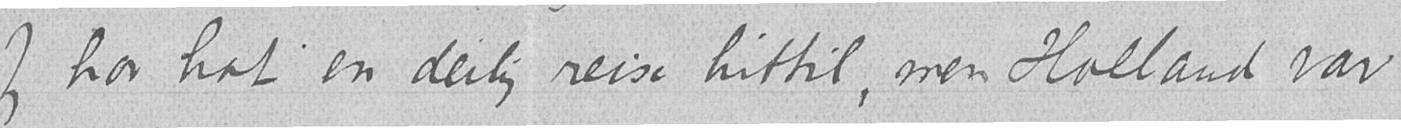Jeg har hat en deilig reise hittil, men Holland var
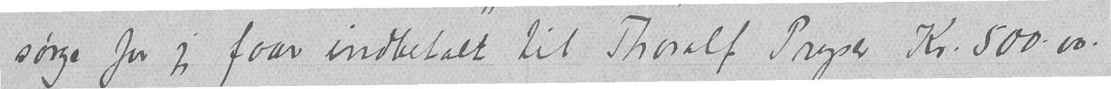sørge for jeg faar indbetalt til Thoralf Pryser Kr. 500.00.
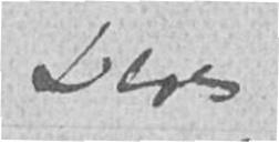Deres
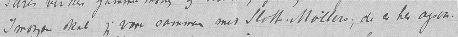Imorgen skal jeg være sammen med Slott-Møllers; de er her ogsaa.
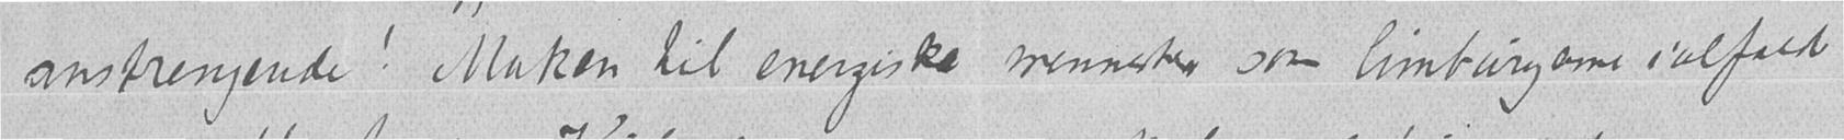anstrengende! Maken til energiske mennesker som limburgerne ialfald
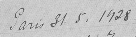Paris 31.5.1928
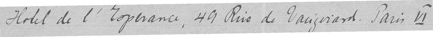Hotel de l'Esperance, 49 Rue de Vaugriard. Paris VI
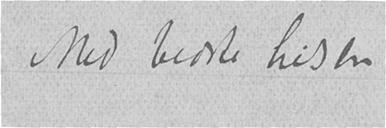Med bedste hilsen
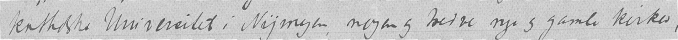katholske Universitet i Nüjmegen, nogen og tredve nye og gamle kirker,
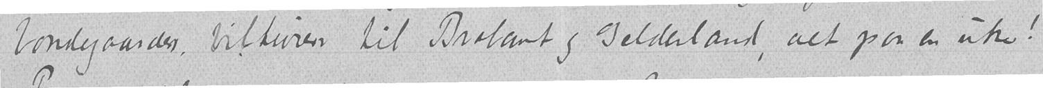bondegaarder, bilturer til Brabant og Gelderland, alt paa en uke!
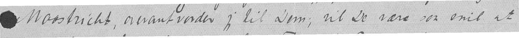Maastricht, overantvorder jeg til Dem; vil De være saa snil at
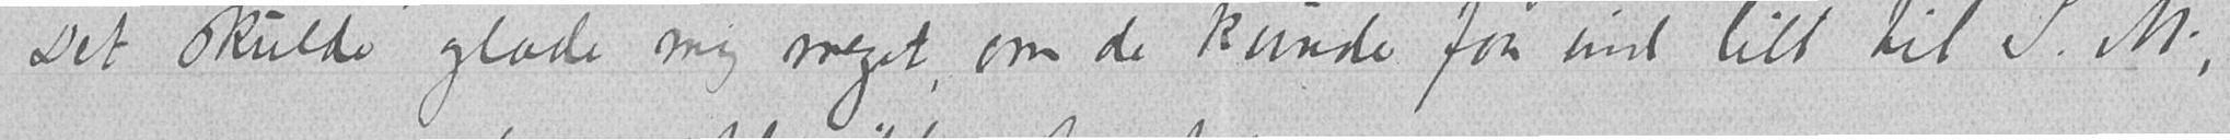Det skulde glæde mig meget, om de kunde faa ind litt til S. M.,
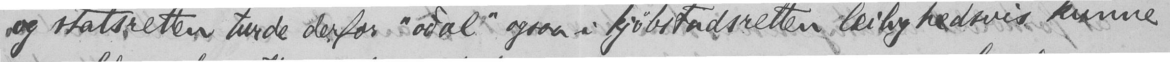og statsretten turde derfor "oðal" ogsaa i kjøbstadsretten leilighedsvis kunne
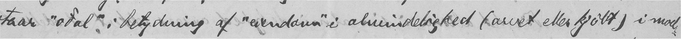staar "oðal" i betydning af "eiendom" i almindelighed (arvet eller kjøbt) i mod-
